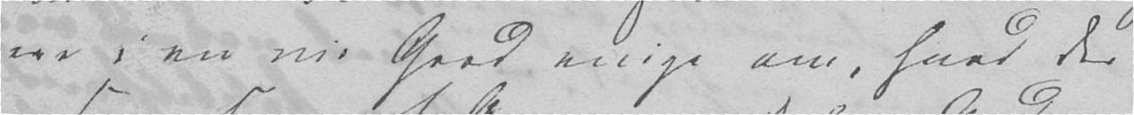ere i en vis Grad enige om, hvad der
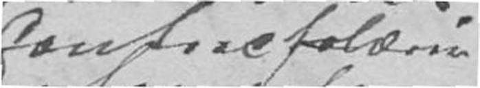tænters folder
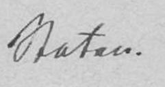Staten.
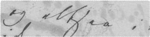og altsaa i
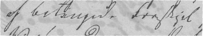af betingede Forskjel
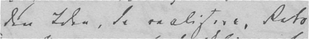den Idee, de realisere, Rets-
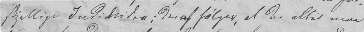skjellige Individer; deraf følger, at der altid maa
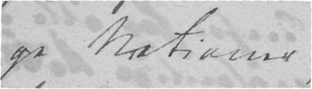ge Nationer
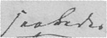saaledes
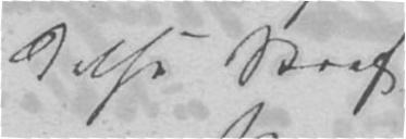delse Straf.
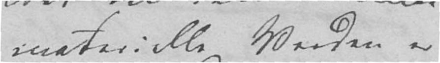materielle Verden er
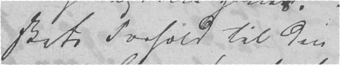skets Forhold til den
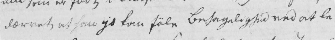dærvet at han jo kan føle Behagelighed ved at le-
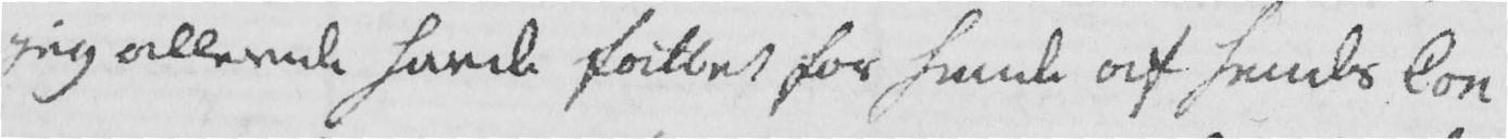jeg allerede havde fattet for hende af hendes Con-
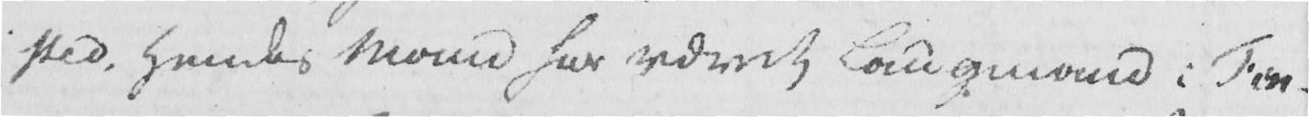sted, hendes Mand har været Laugmand i Fin-
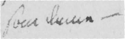som denne -
